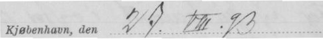Kjøbenhavn, den 27. VIII. 93
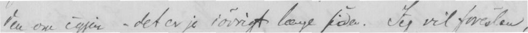Dem om igjen; det er jo iøvrigt længe siden. Jeg vil foreslaa,
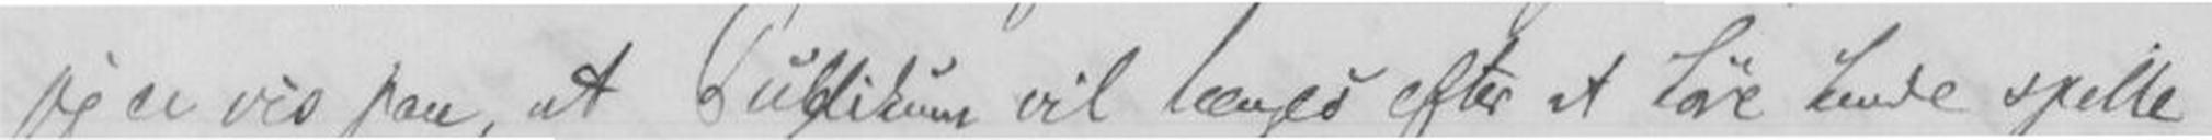jeg er vis paa, at Publikum vil længes efter at høre hende spille
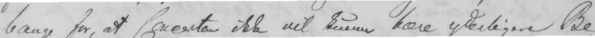bange for, at Concerten ikke vil kunne bære yderligere Be
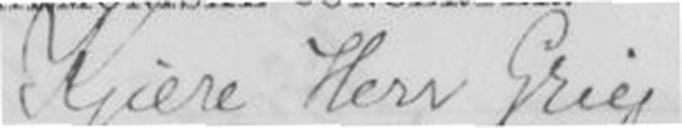Kjære Herr Grieg !
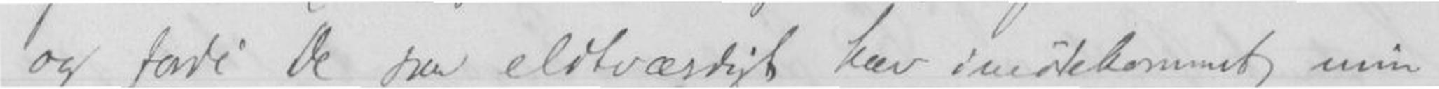og fordi De saa elskværdigt har imøtekommet min
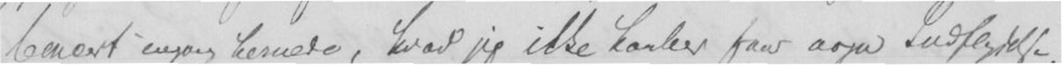Concert engang hernede, hvad jeg ikke tænker faa ;
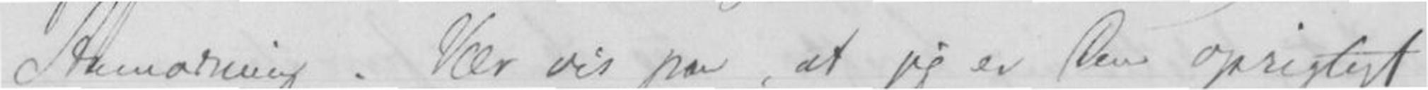Anmodning. Vær vis paa, at jeg er Dem oprigtigt
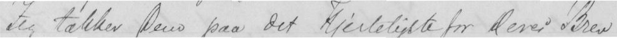Jeg takker Deres paa det hjerteligste for Deres Brev
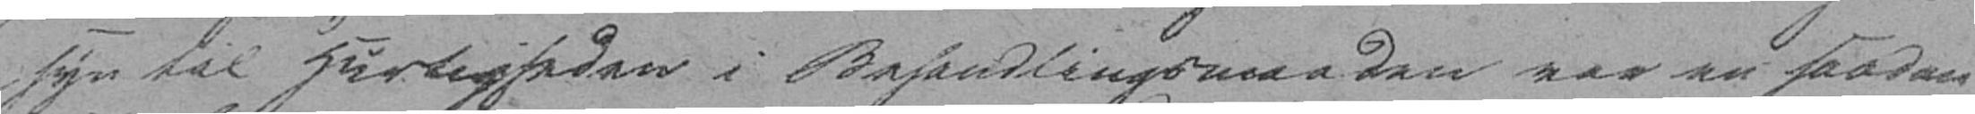syn til Hurtigheden i Behandlingsmaaden var en saadan
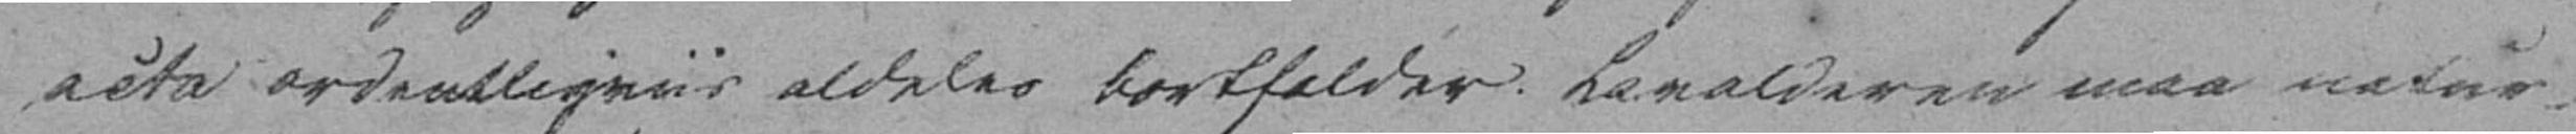acta ordentligviis aldeles bortfalder. Lavalderen maa natur-
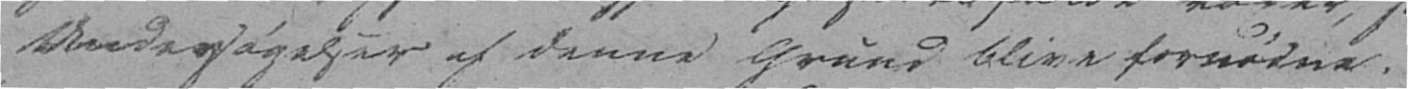Undersøgelser af denne Grund blive fornødne.
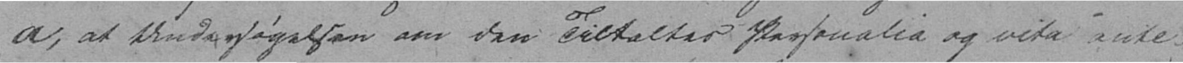a, at Undtagelsen om den Tiltaltes Personalia og vite ante
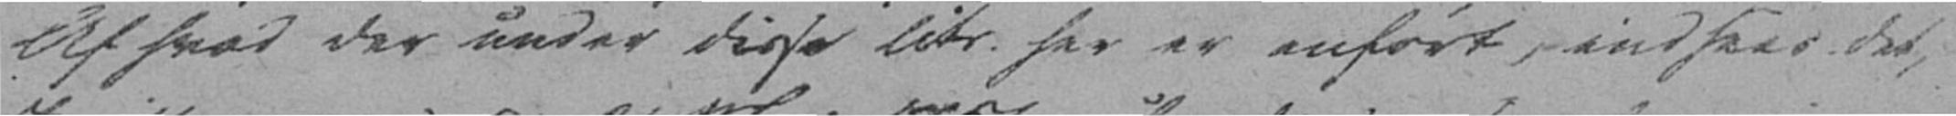Af hvad der under disse litr. her er anført, indsees det,
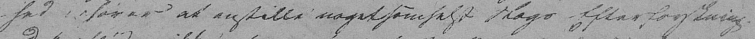hed, anfører at anstille nogetsomhelst Slags Efterforskning.
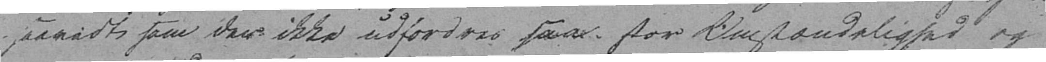saavidt som der ikke udfordres saa stor Omstændelighed og
